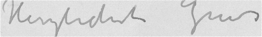Herzlichste Grus
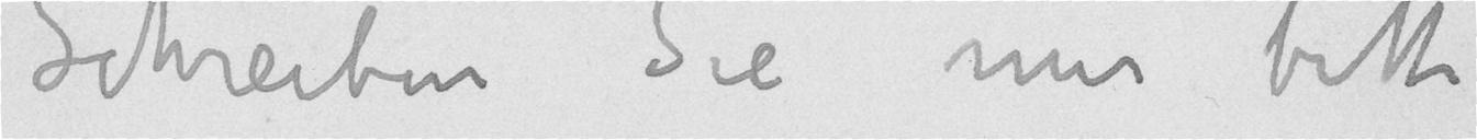Schreiben Sie mir bitte
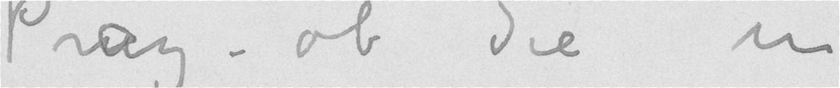Prag – ob Sie in
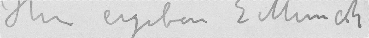Ihr ergeben E Munch
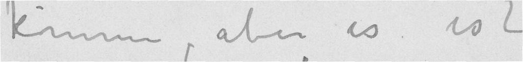kömmen, aber es ist
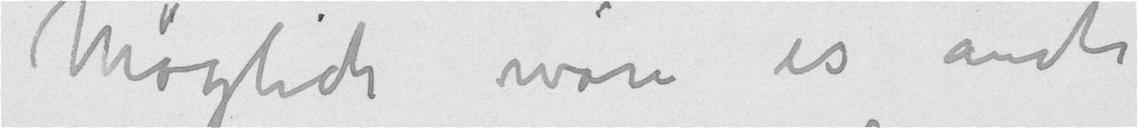Möglich wäre es auch
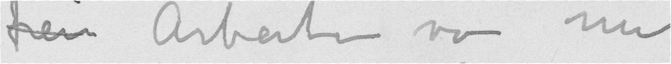freie Arbeiten von mir
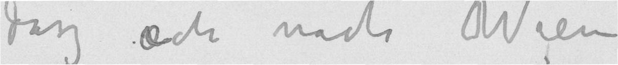dasz ich nach Wien
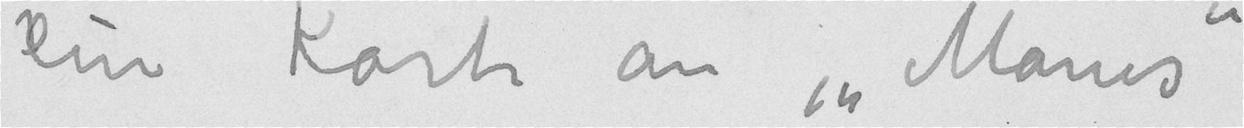ein Karte an «Manes»
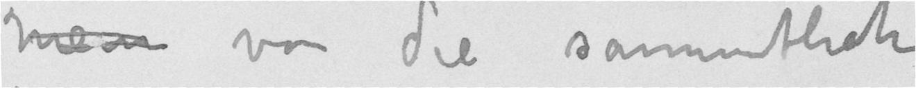mein von die sammtliche
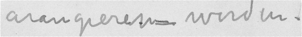arangieren werden.
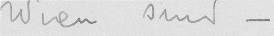Wien sind –
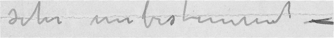sehr unbestimmt –
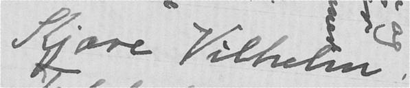Kjære Vilhelm.
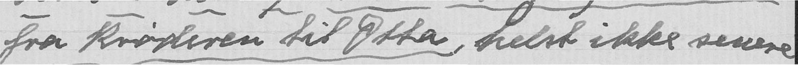fra Krøderen til Otta, helst ikke senere
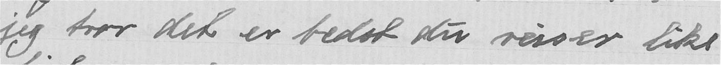jeg tror det er bedst du reiser like
